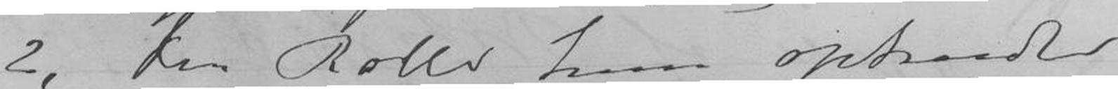2, den Rolle hun optræder
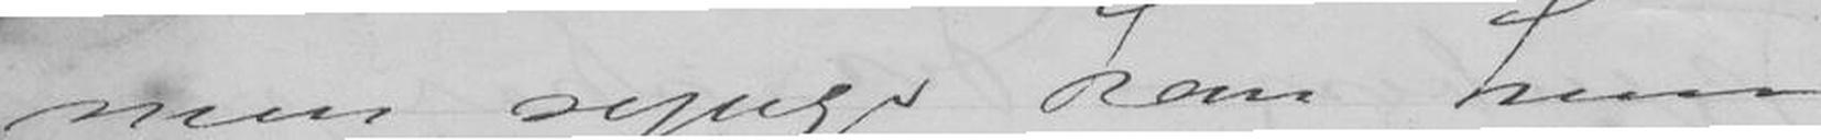men synge kan hun
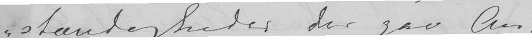stændigheder der gav An-
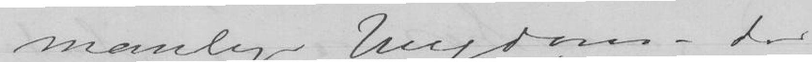manlige Ungdom - der
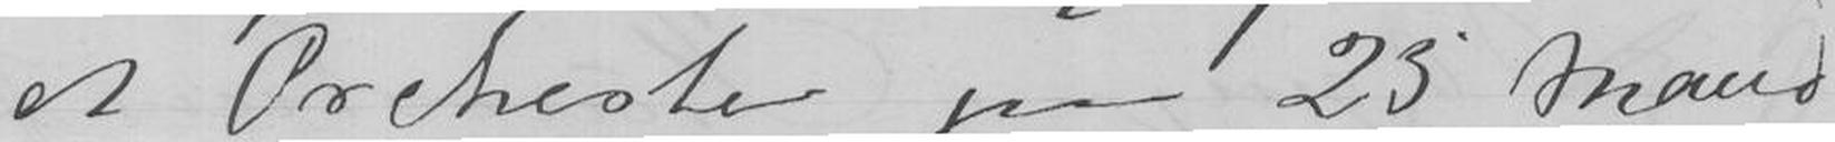et Orchester paa 25 Mand
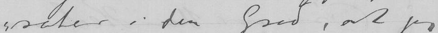rater i den Grad, at jeg
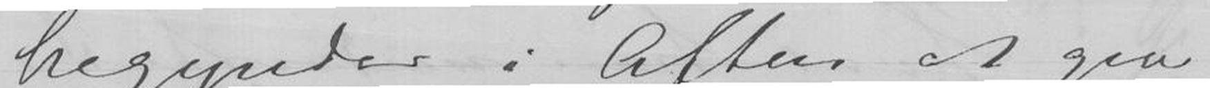begynder i Aften at give
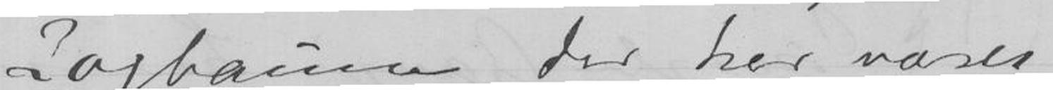Zagbauen der har været
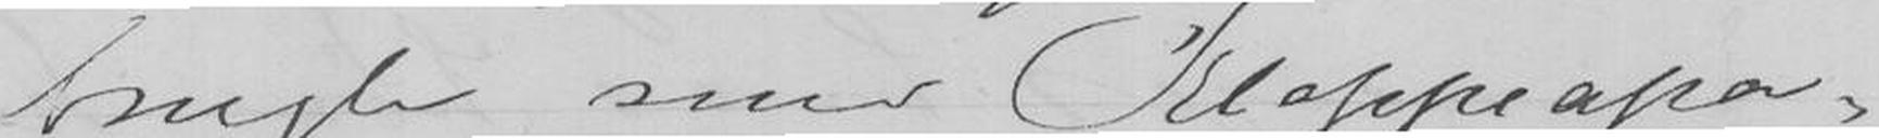brugte sine Klappeapa-
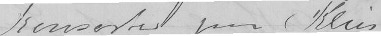Konserter paa Klin
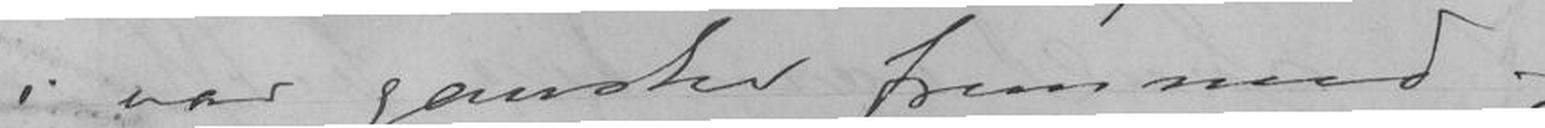i var ganske fremmed?
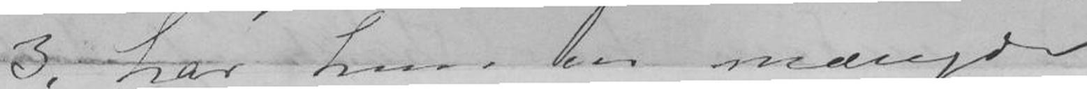3, har hun en mængde
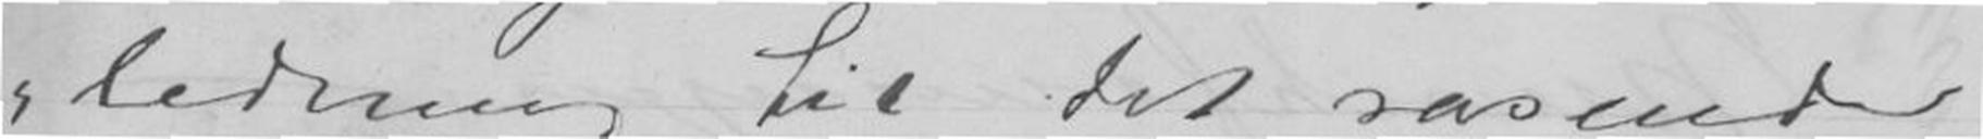ledning til det rasende
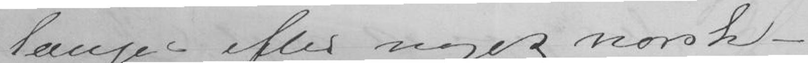længes efter noget norsk -
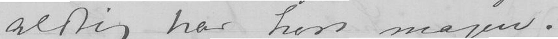aldrig har hørt magen.
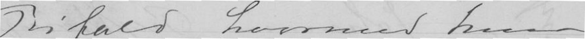Bifald hvormed hun
